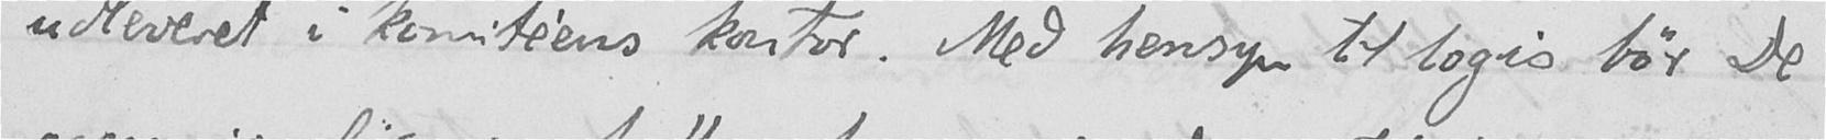udleveret i komitéens kontor. Med hensyn til logis bør De
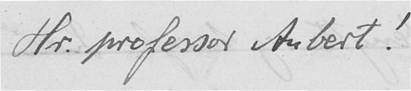Hr. professor Aubert!
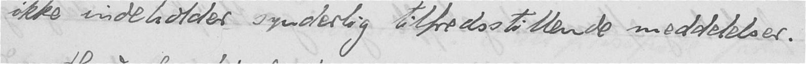ikke indeholder synderlig tilfredsstillende medddelser.
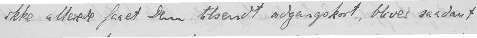ikke allerede faaet Dem tilsendt adgangskort, bliver saadant
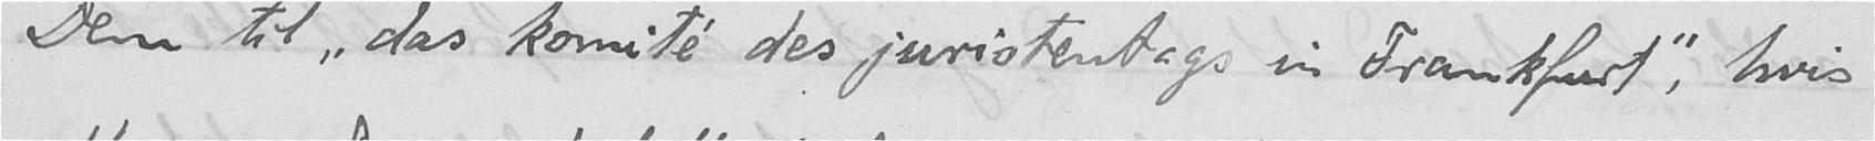Dem til "das komité des juristentags in Frankfurt", hvis
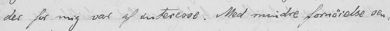der for mig var af interesse. Med mindre fornøielse sen-
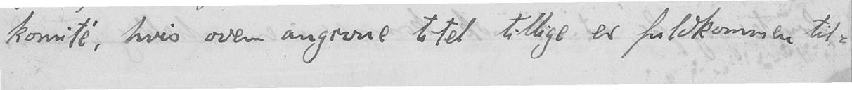komité, hvis oven angivne titel tillige er fuldkommen til-
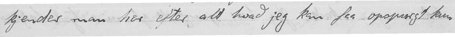kjender man her efter alt hvad jeg kan faa opspurgt kun
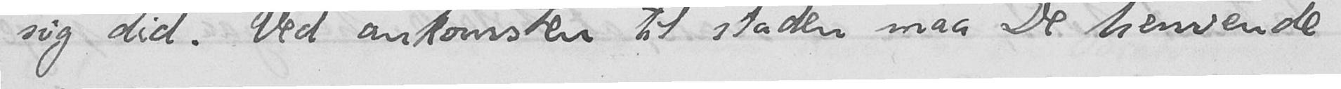sig did. Ved ankomsten til staden maa De henvende
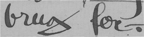brag for.
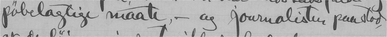pøbelagtige maate, - og Journalisten paastod,
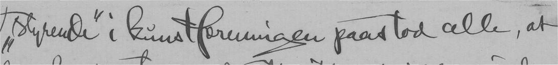"styrende" i kunstforningen paastod alle, at
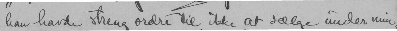han havde streng ordre til ikke at sælge under min,
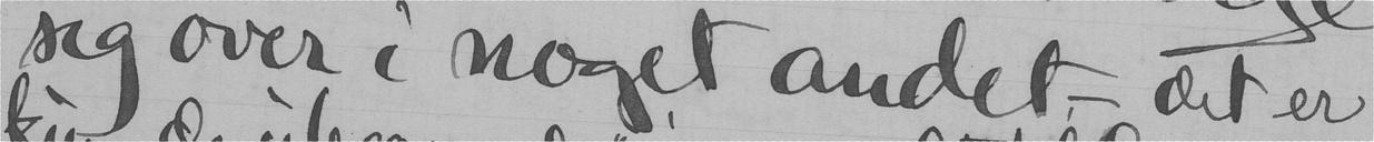sig over i noget andet, - det er
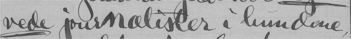vede journalister i hundene,
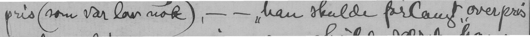pris (som var lav nok), -- "han skulde forlangt "overpris"
